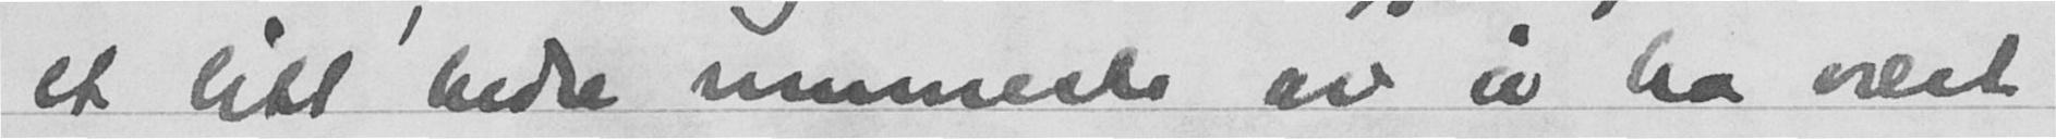et litt bedre menneske av å ha vært
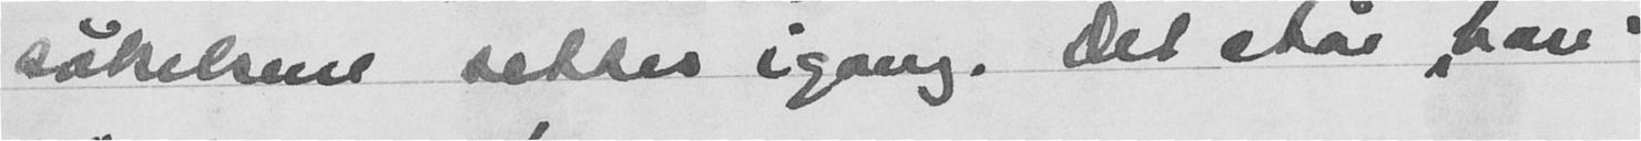søkelsene settes igang. Det står "han"
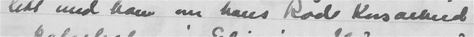litt med ham om hans Røde Kors arbeid
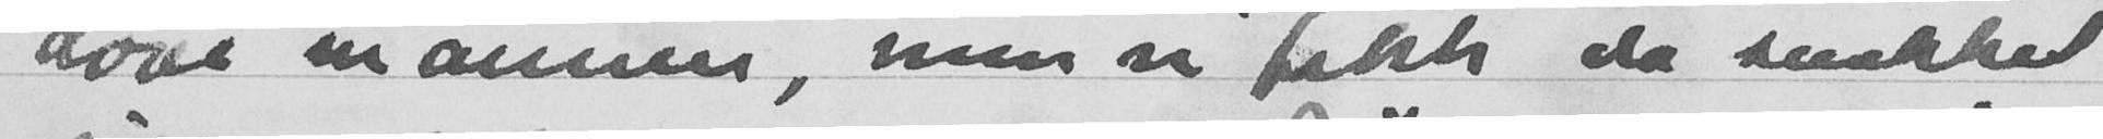døve mannen, men vi fikk da snakket
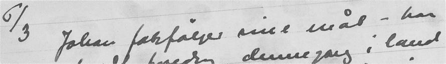6/3 Johan forfølger sine mål - har
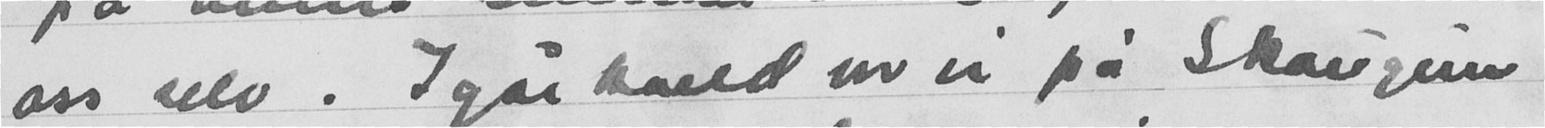oss selv. Igårkveld var vi på Skaugum
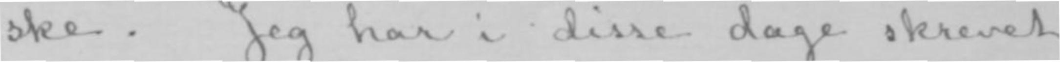ske. Jeg har i disse dage skrevet
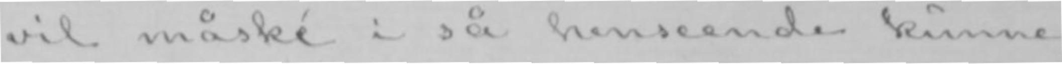vil måské i så henseende kunne
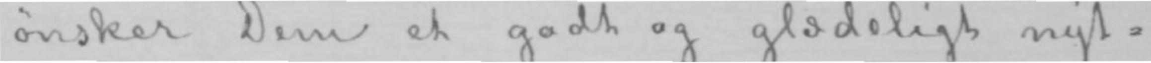ønsker Dem et godt og glædeligt nyt-
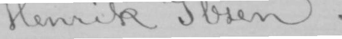Henrik Ibsen.
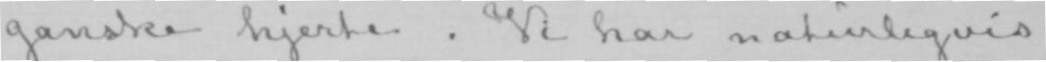ganske hjerte. Vi har naturligvis
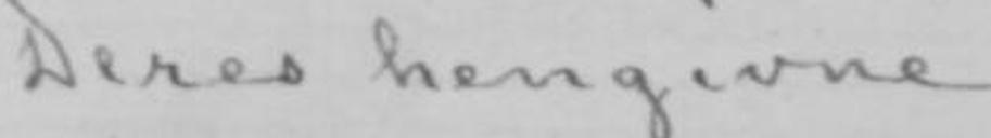Deres hengivne
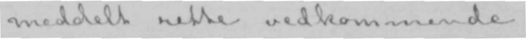meddelt rette vedkommende.-
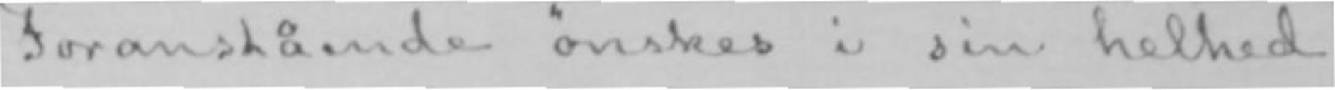Foranstående ønskes i sin helhed
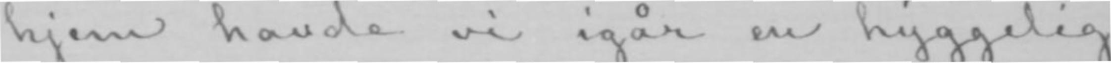hjem havde vi igår en hyggelig
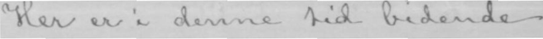Her er i denne tid bidende
